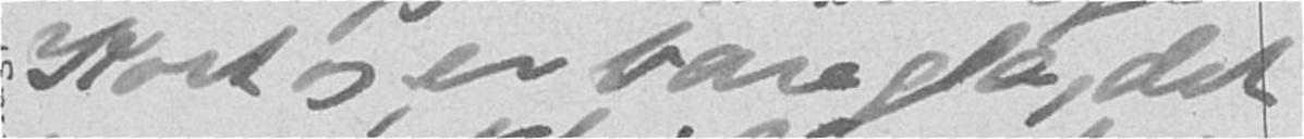Kort og er bare gla, det
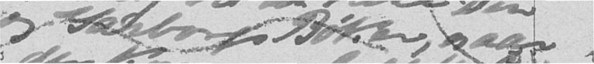og Garborgs Bøker, naar
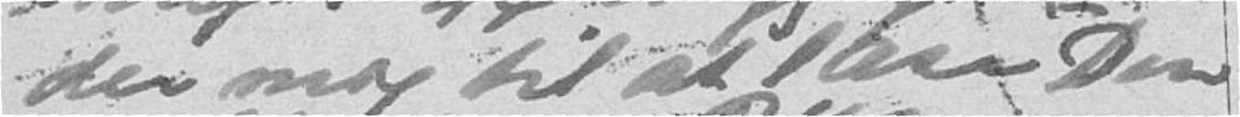der mig til at læse Din
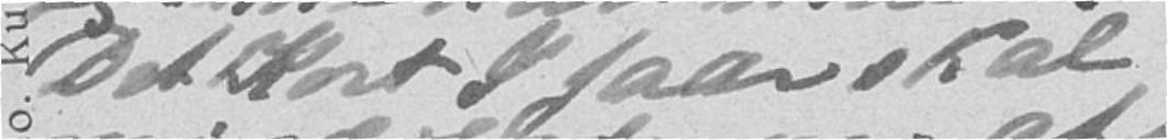Det Kort I faar skal
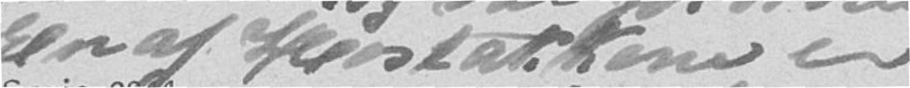En af Høstakkene er
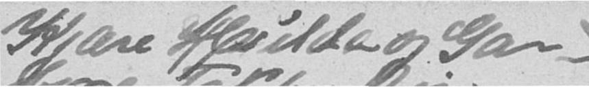Kjære Hulda og Gar-
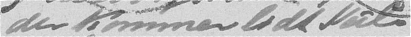der kommer lidt Jule-
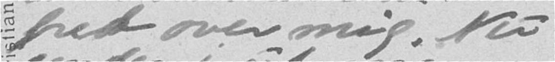fred over mig. Nu
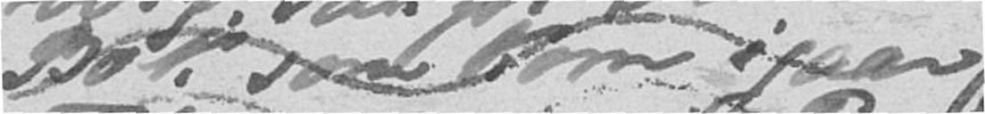Bok som kom igaar
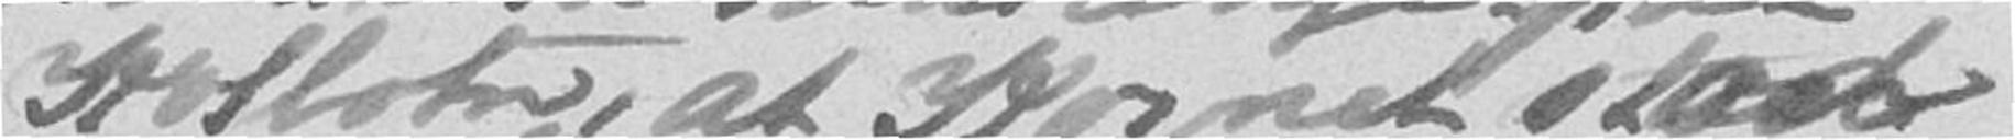Kolbotn, at Kornet stod
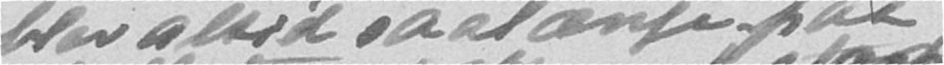blev altid saalænge paa
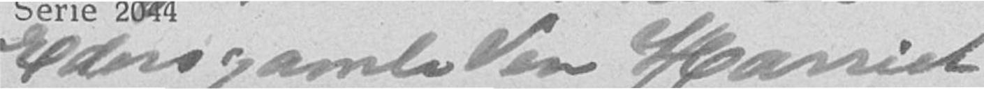Eders gamle Ven Harriet
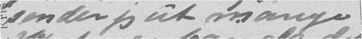sender jeg ut mange
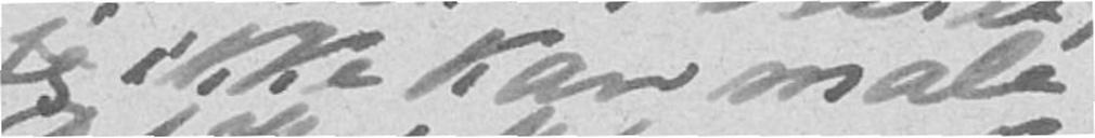jeg ikke kan male.
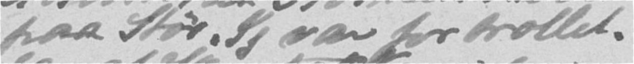paa Stør. Jeg var fortrollet.
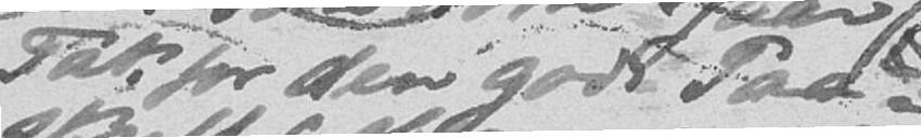Tak for den godt Paa-
